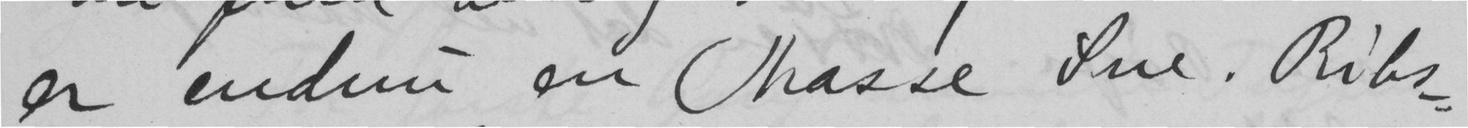er endnu en Masse Sne. Ribs-
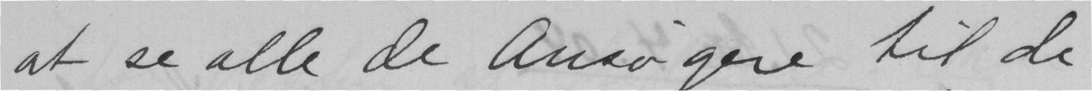at se alle de Ansøgere til de
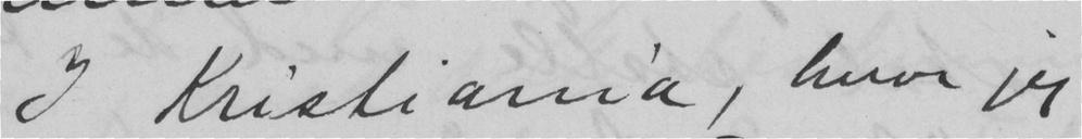I Kristiania, hvor jeg
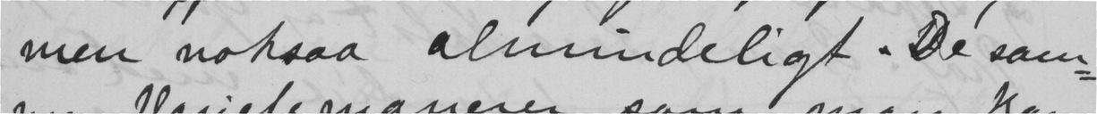men noksaa almindeligt. De sam-
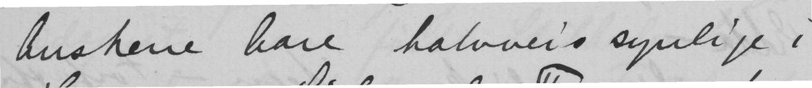buskene bare halvveis synlige i
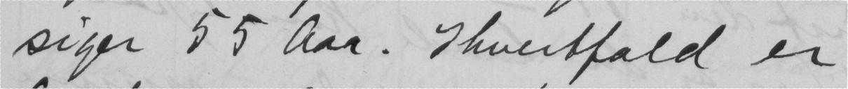siger 55 Aar. I hvertfald er
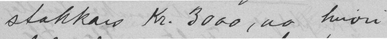stakkars Kr. 3000, 00 hvori
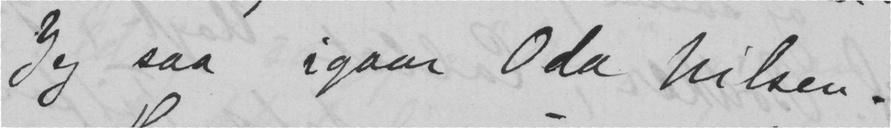Jeg saa igaar Oda Nilsen.
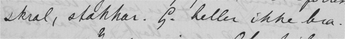skral, stakkar. G. heller ikke bra.
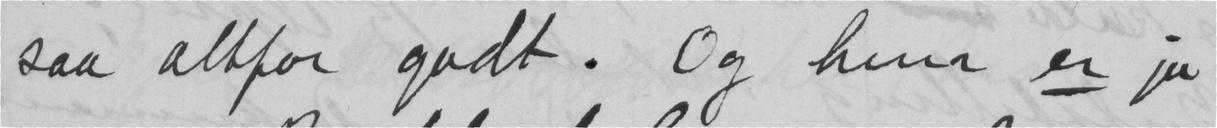saa altfor godt. Og hun er jo
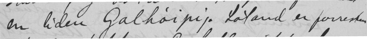en liden Galhøipig. Løland er forresten
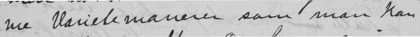me Varietemanerer som man kan
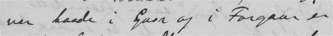var baade i Gaar og i Forgaar er
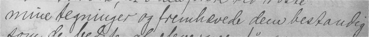mine tegninger og fremhævede dem bestandig
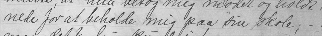nede for at beholde mig paa sin skole; -
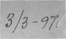3/3-97.
